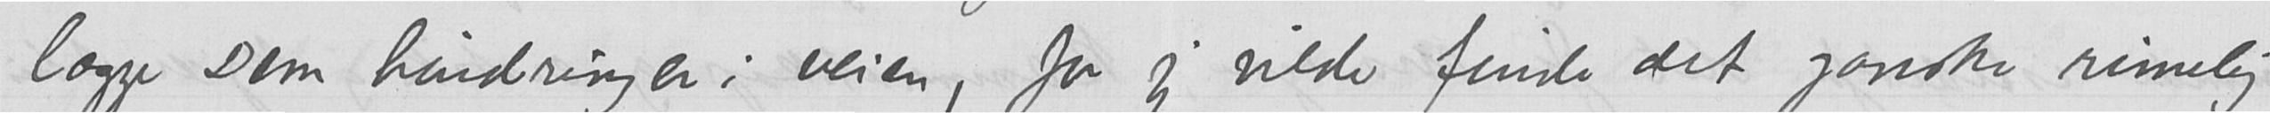lægge Dem hindringer i veien, for jeg vilde finde det ganske rimelig
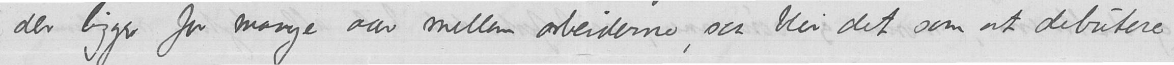der ligger for mange aar mellem arbeiderne, saa blir det som at debutere
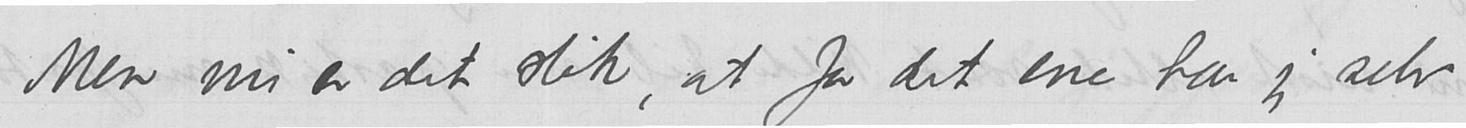Men nu er det slik, at for det ene har jeg selv
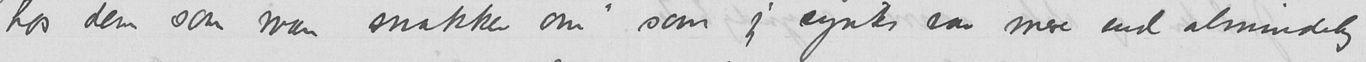hos dem som man snakker om" som jeg syntes var mere end almindelig
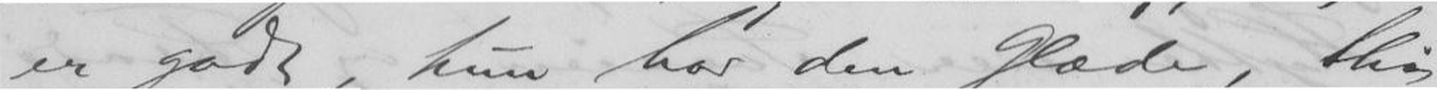er godt, hun har den Glæde, thi
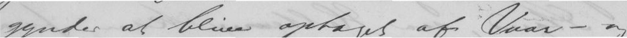gynder at blive optaget af Vaar- og
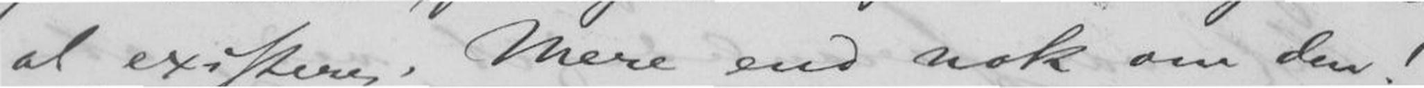at existere. Mere end nok om den!
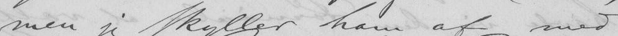men jeg skylder ham af med
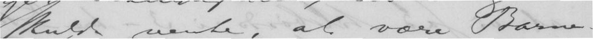kulde vente, at være Barne-
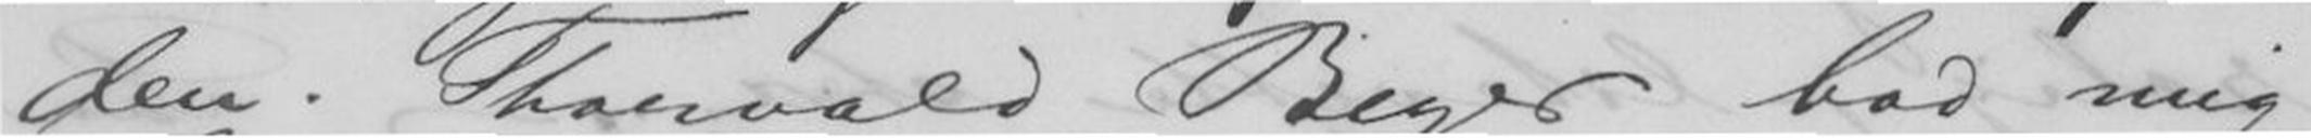den. Thorvald Beyer bad mig
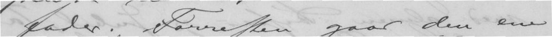fader. Forresten gaar den ene
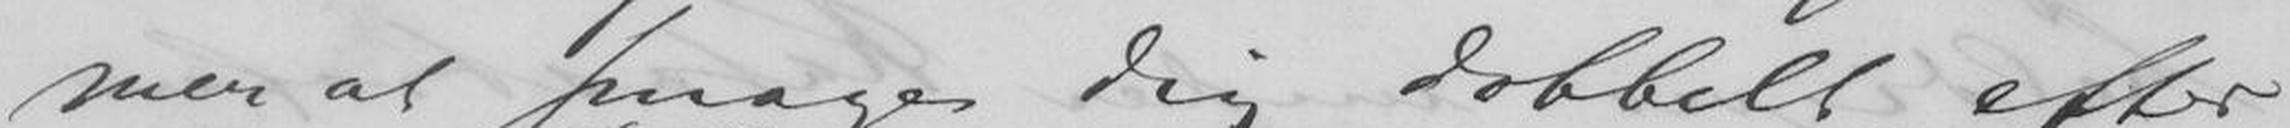mer at smage dig dobbelt efter
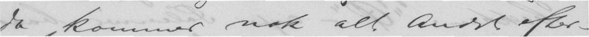da kommer nok alt Andet efter-
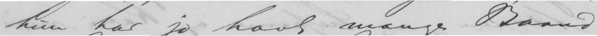hun har jo havt mange Baand
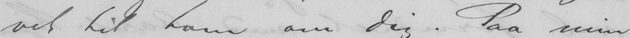vet til ham om dig. Paa min
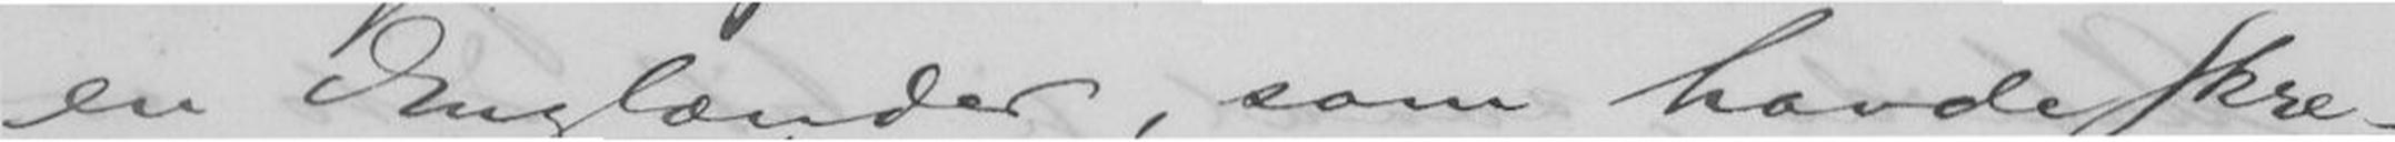en Englænder, som havde skre-
